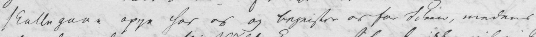skulle gaae oppe hos os og begeistre os for Ideen, medens
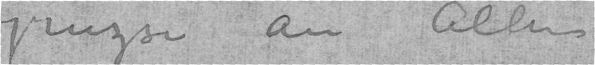Gruzse an Allen
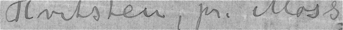Hvitsten, pr. Moss
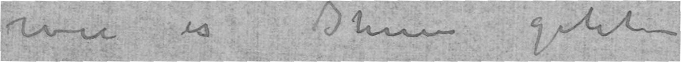wie es Ihnen geht
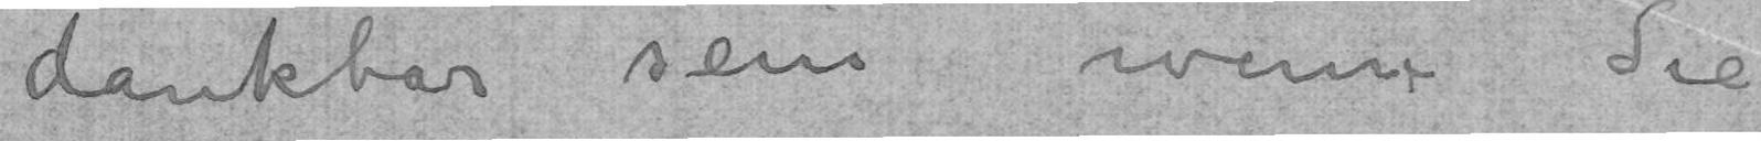dankbar sein wenn Sie
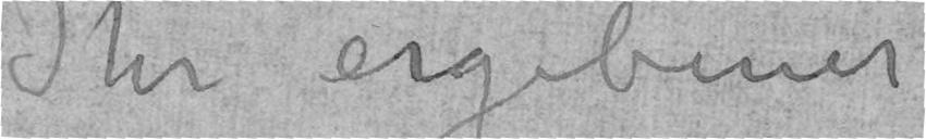Ihr ergebener
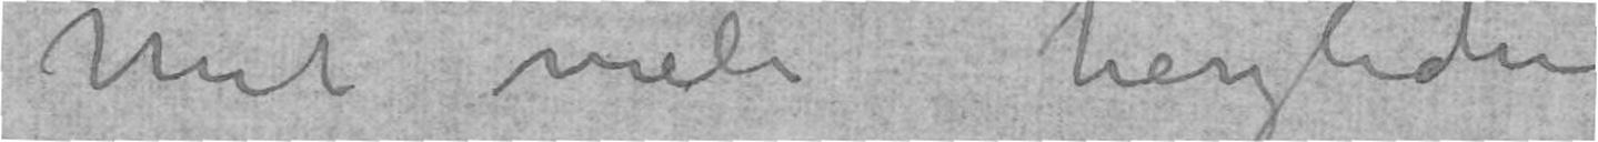Mit viele herzlichen
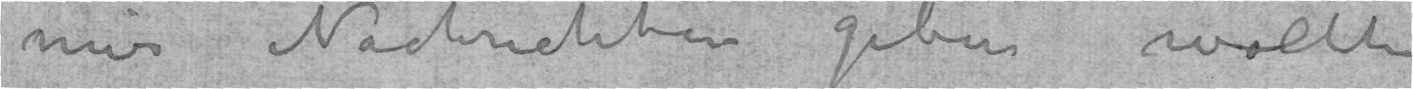mir Nachrichten geben wollte
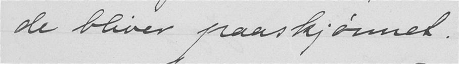de bliver paaskjønnet.
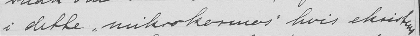i dette "mikrokosmos" hvis eksistens
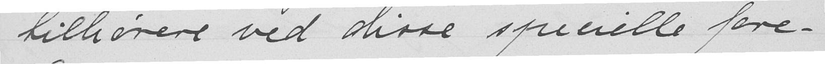tilhørere ved disse specielle fore-
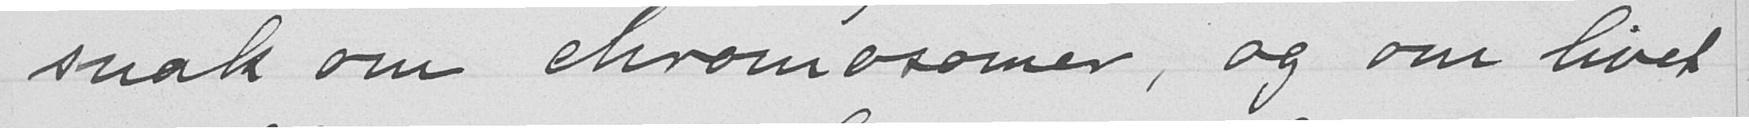snak om chromosomer, og om livet
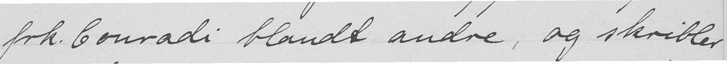frk. Conradi blandt andre, og skribler
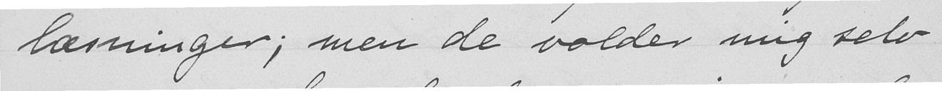læsninger; men de volder mig selv
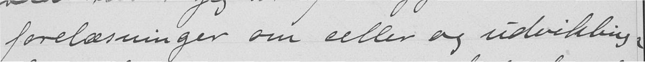forelæsninger om celler og udvikling
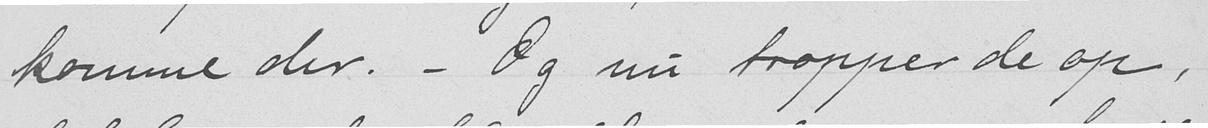komme der. - Og nu tropper de op,
